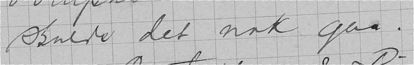skulde det nok gaa.
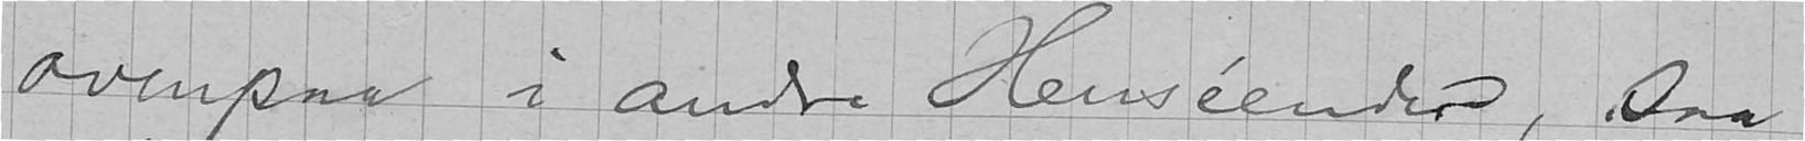ovenpaa i andre Henseender, saa
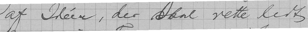af Ideer, der skal rette lidt
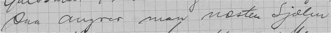saa angrer man næsten Sjælen
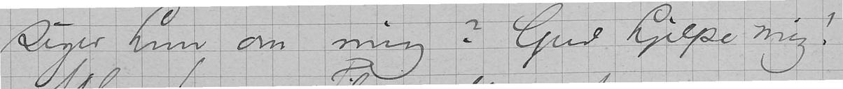siger hun om mig? Gud hjelpe mig!
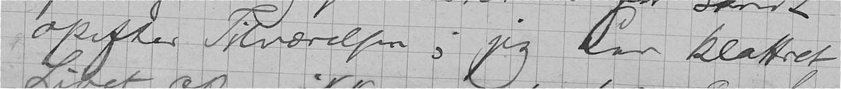opefter Tilværelsen; jeg har klattret
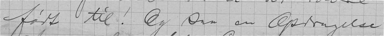født til! Og saa en Opdragelse
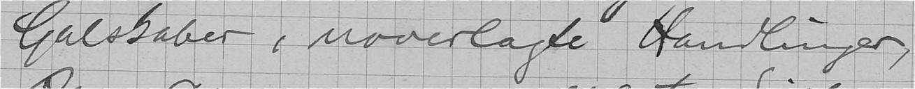Galskaber, uoverlagte Handlinger,
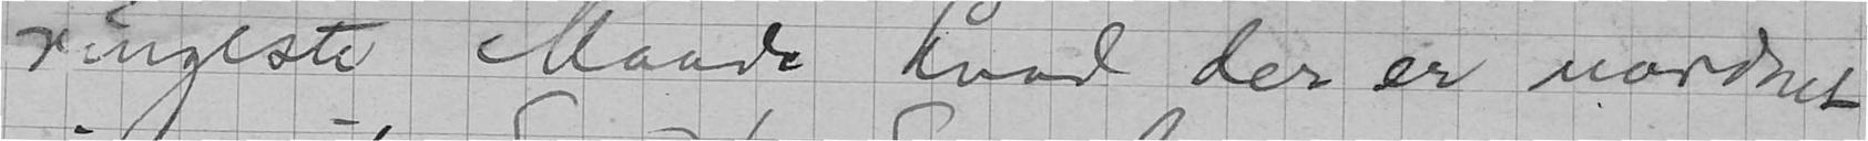ringeste Maade hvad der er uordnet
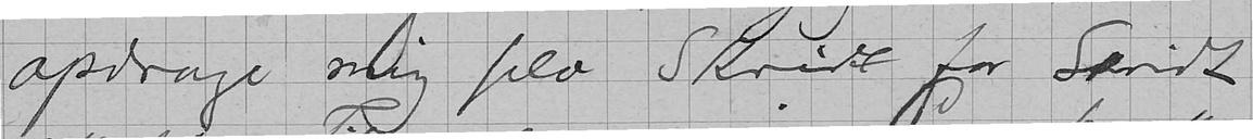opdrage mig selv Skridt for Skridt
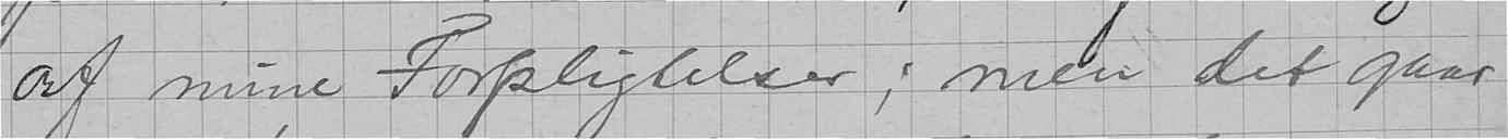af mine Forpligtelser; men det gaar
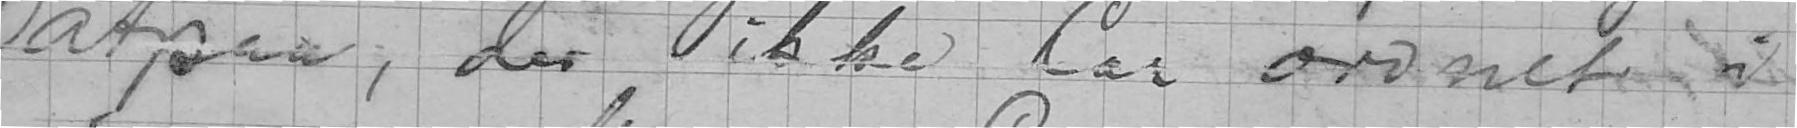atpaa, der ikke har ordnet i
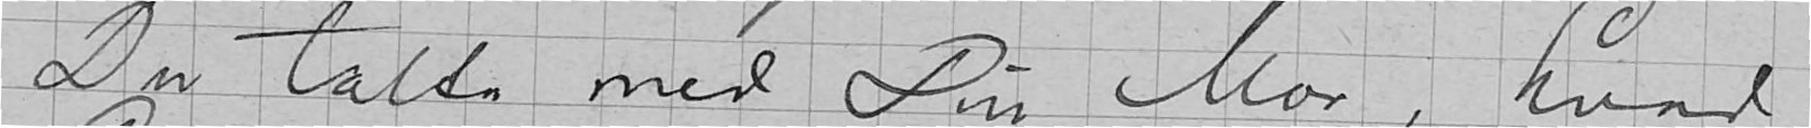Du talte med Din Mor, hvad
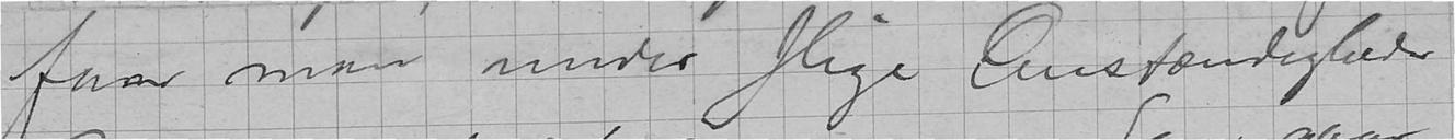faar man under slige Omstændigheder
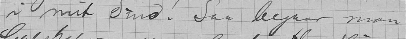i mit Sind! Saa begaar man
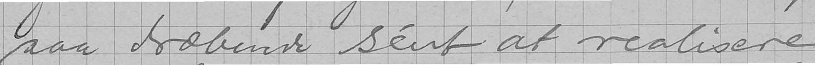saa dræbende sent at realisere
